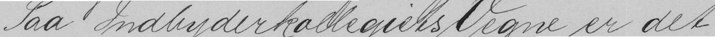Paa Indbyderkollegiets Vegne er det
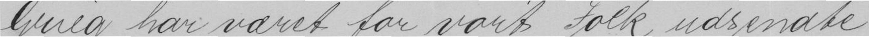Grieg har været for vort Folk udsendte
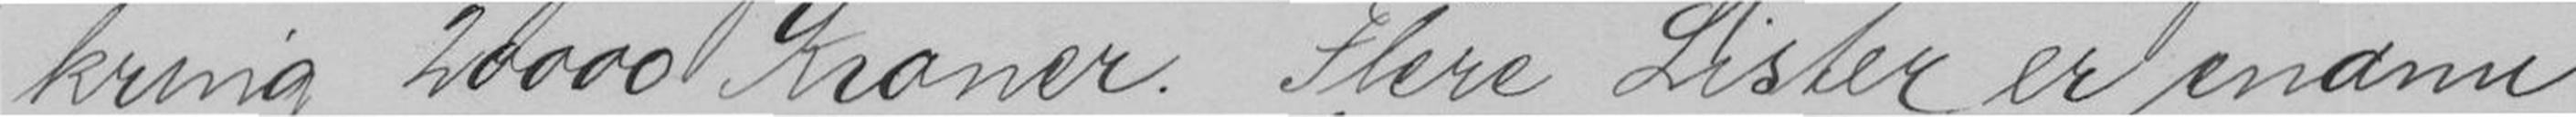kring 20000 Kroner. Flere Lister er endnu
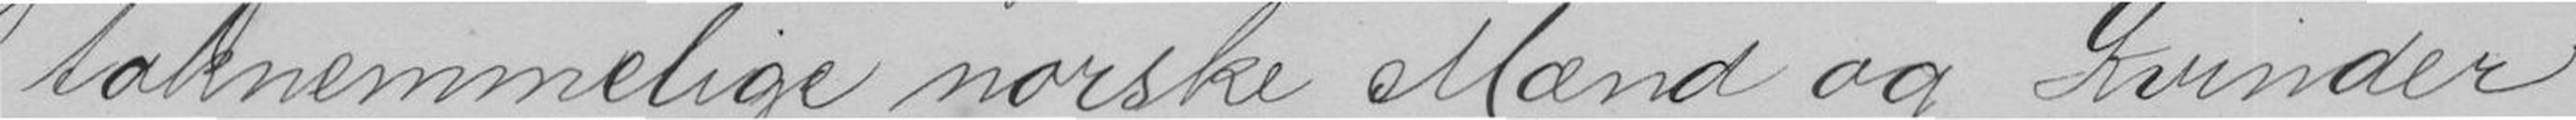taknemmelige norske Mænd og Kvinder
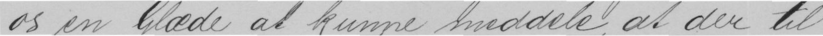os en Glæde at kunne meddele, at der til
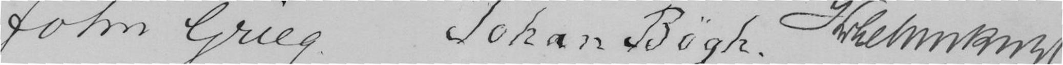John Grieg Johan Bøgh. H Lehmkuhl
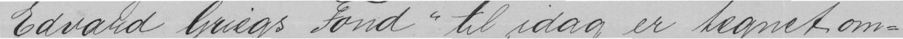Edvard Griegs Fond til idag er tegnet om-
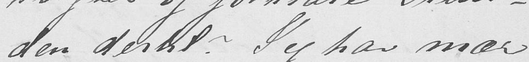den dertil? Jeg har mær-
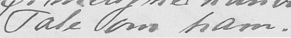Tale om ham.
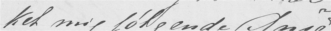ket mig følgende Ansø-
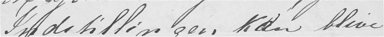Indstillingen kan blive
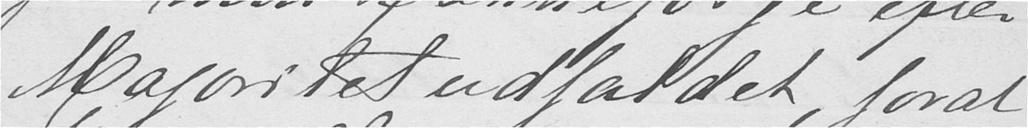Majoritetudfaldet, forat
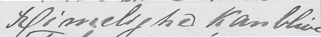Rimelighed kan blive
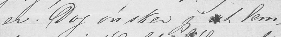er. Dog ønsker jeg at lem-
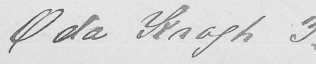Oda Krogh 3.
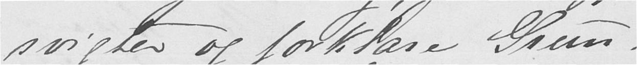svigter og forklare Grun-
